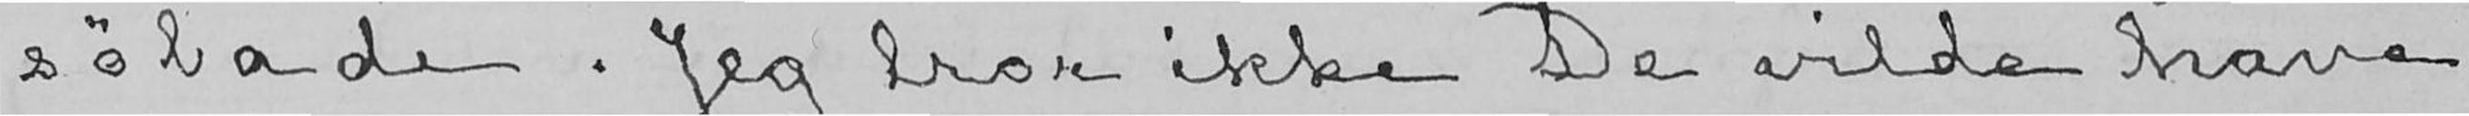søbade. Jeg tror ikke De vilde have
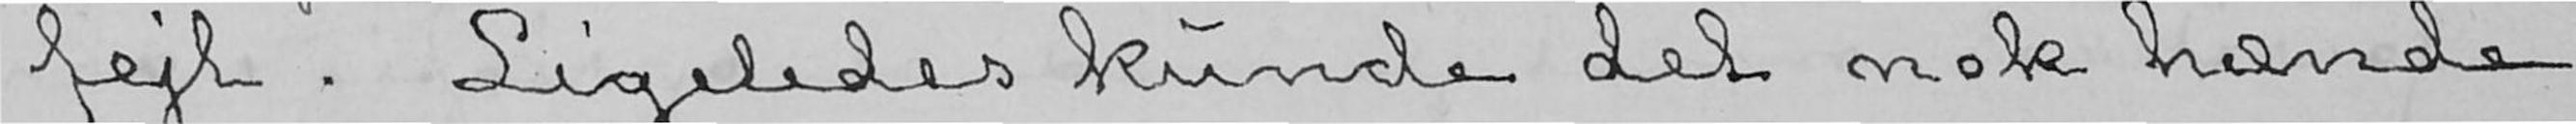fejl. Ligeledes kunde det nok hænde
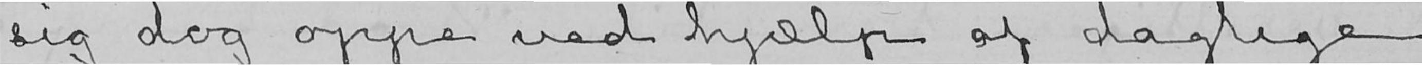sig dog oppe ved hjælp af daglige
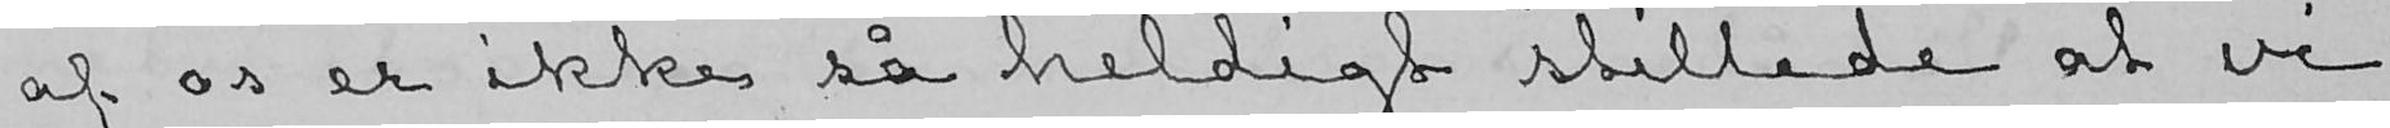af os er ikke så heldigt stillede at vi
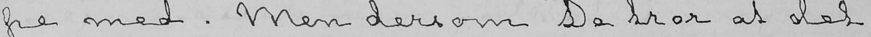pe med. Men dersom De tror at det
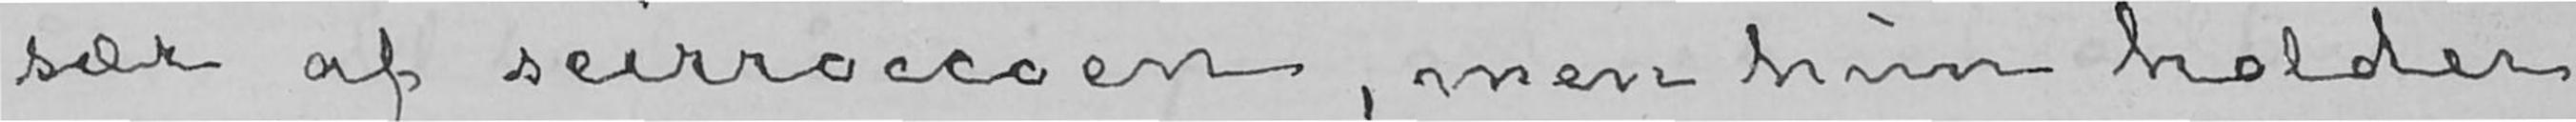sær af scirroccoen, men hun holder
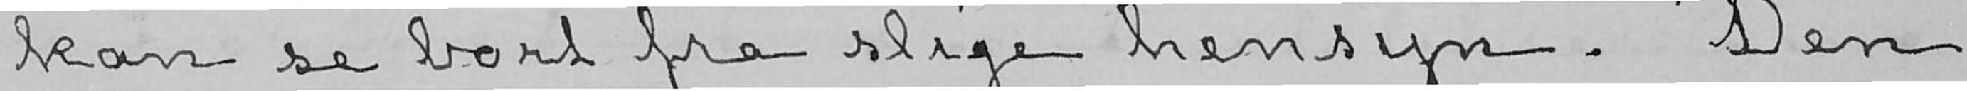kan se bort fra slige hensyn. Den
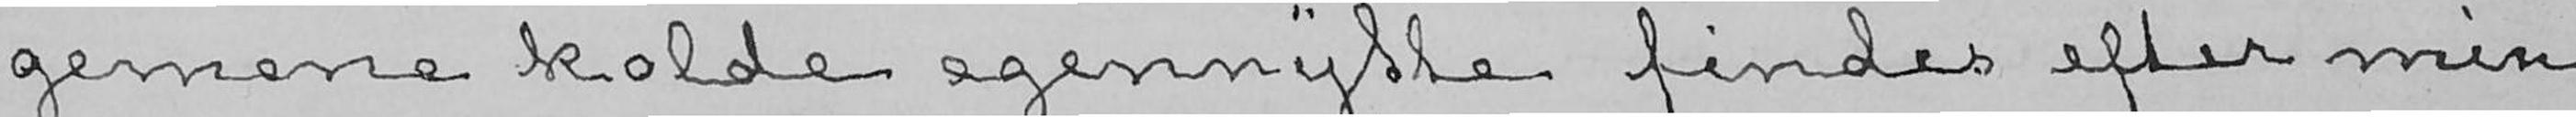gemene kolde egennytte findes efter min
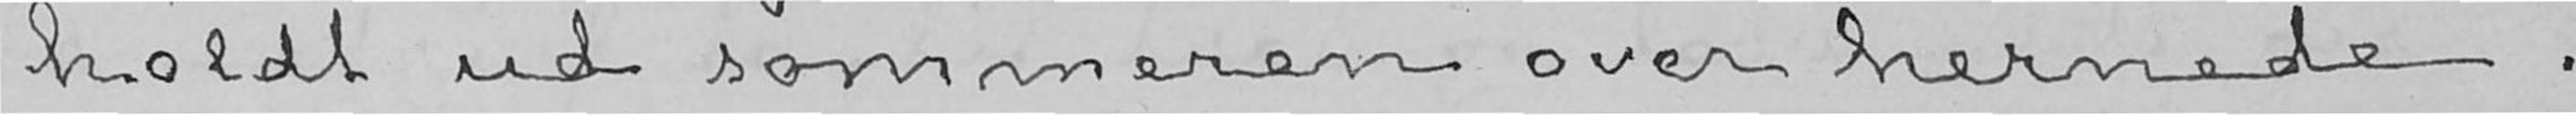holdt ud sommeren over hernede.
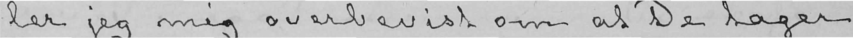ler jeg mig overbevist om at De tager
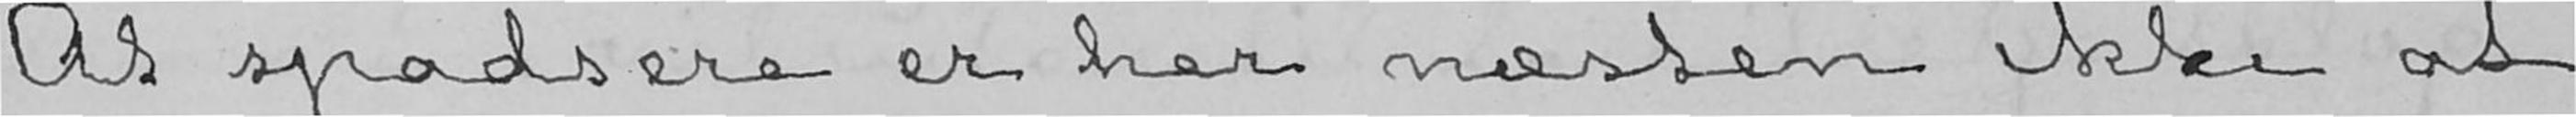At spadsere er her næsten ikke at
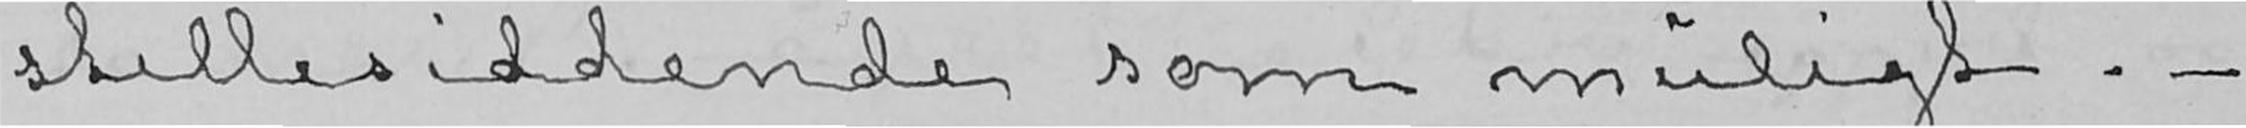stillesiddende som muligt.-
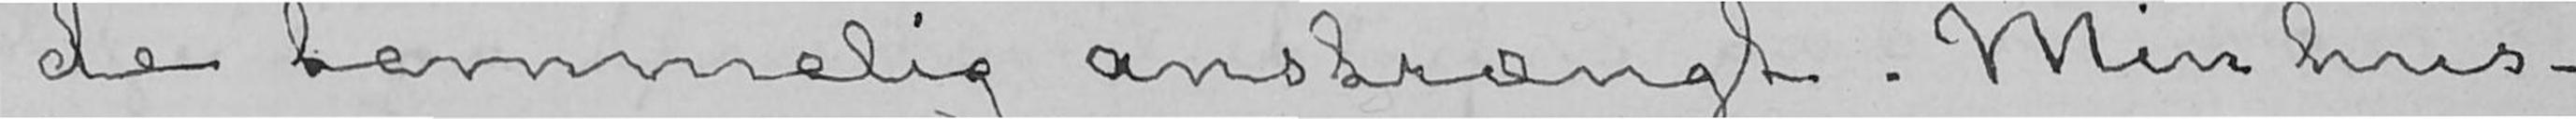de temmelig anstrængt. Min hus-
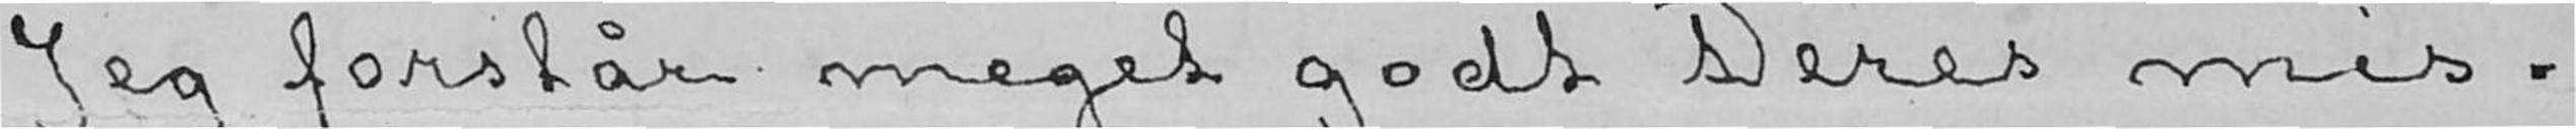Jeg forstår meget godt Deres mis-
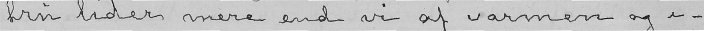tru lider mere end vi af varmen og i-
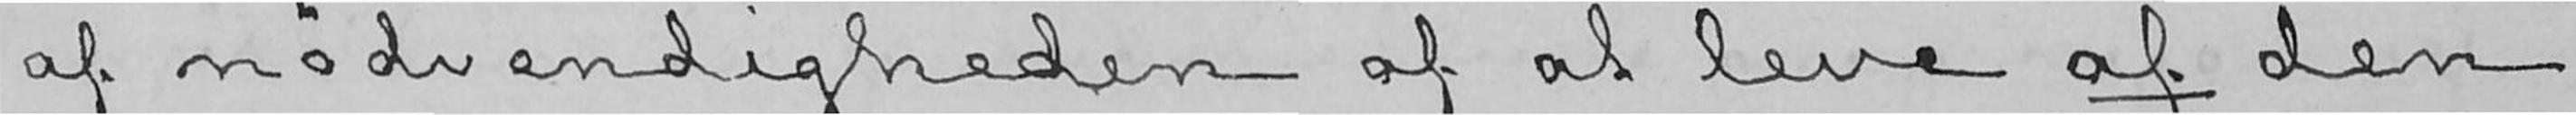af nødvendigheden af at leve af den
